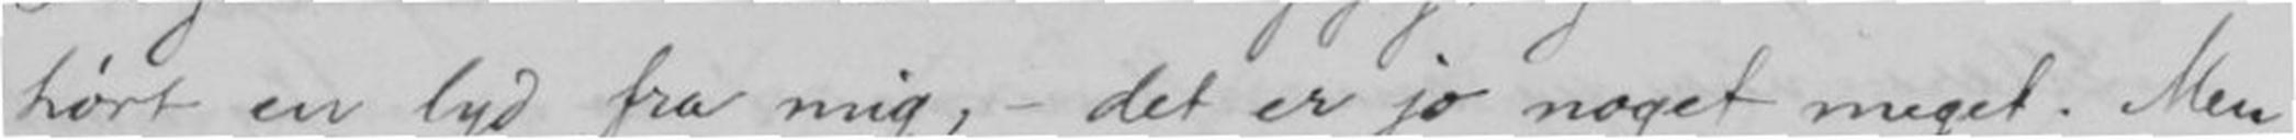hørt en lyd fra mig, - det er jo noget meget. Men
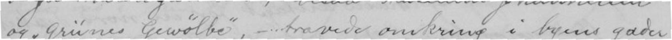og "grünes Gewölbe", - travede omkring i byens gader
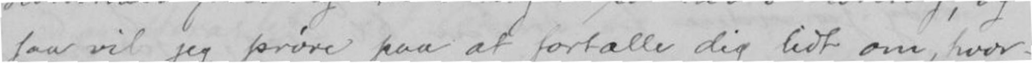saa vil jeg prøve paa at fortælle dig lidt om, hvor-
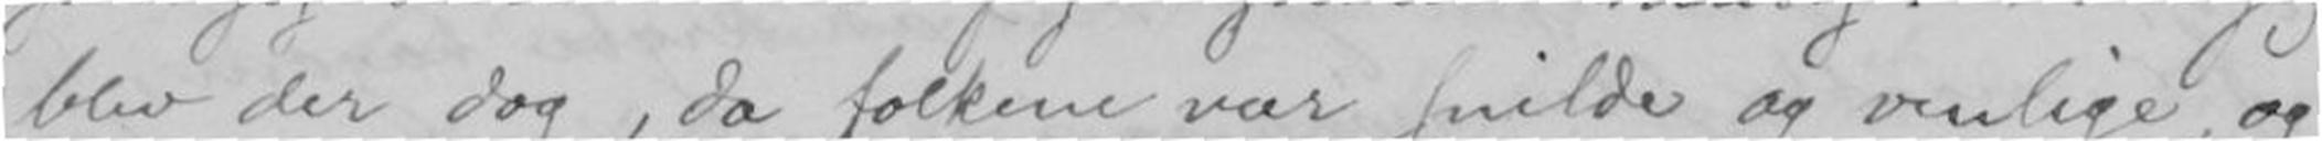blev der dog, da folkene var snilde og venlige, og
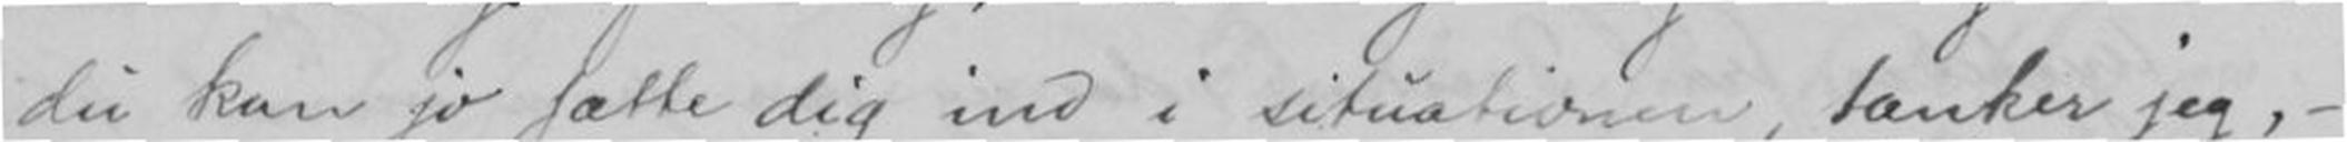du kan jo sætte dig ind i situationen, tænker jeg, -
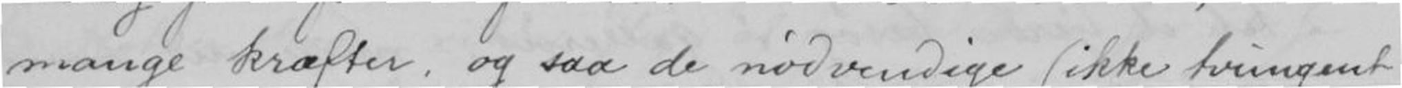mange kræfter og saa de nødvendige (ikke tvunget
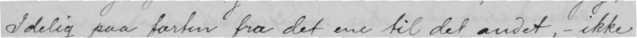Idelig paa farten fra det ene til det andet, - ikke
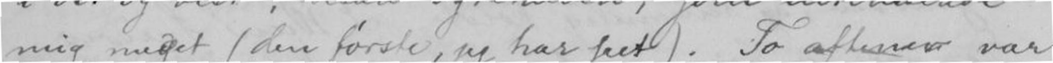mig meget (den første jeg har seet). To aftener var
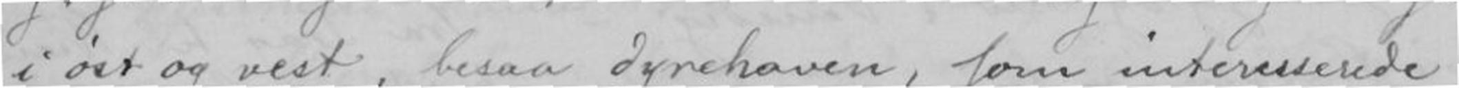i øst og vest, besaa dyrehaven, som interesserede
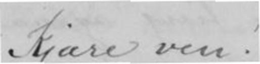Kjære ven!
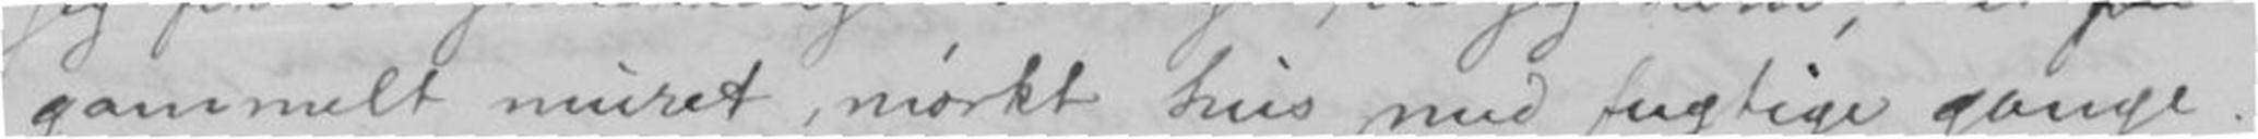gammelt muret mørkt hus med fugtige gange
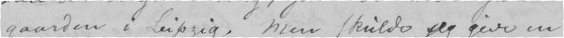gaarden i Leipzig. Men skulde jeg give en
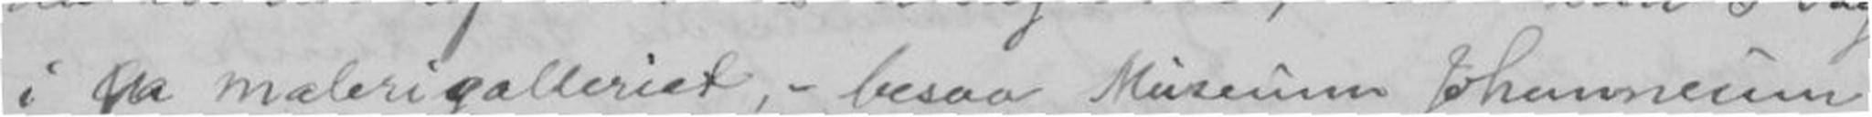i malerigalleriet,- besaa Mùseùm Johawnerum
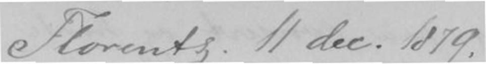Florentz. 11 dec. 1879.
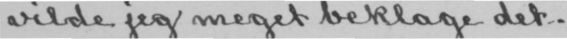vilde jeg meget beklage det.
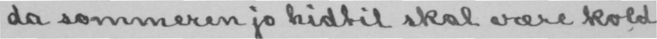da sommeren jo hidtil skal være kold
